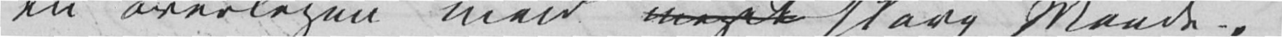en overlegen men meget skarp Maade,
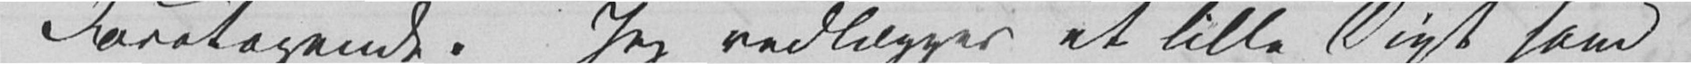Foretagende. Jeg vedlægger et lille Digt som
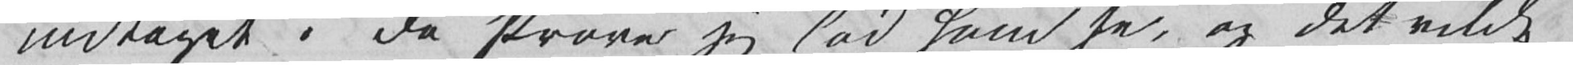indtaget i de Prøver jeg lod ham se, og det vilde
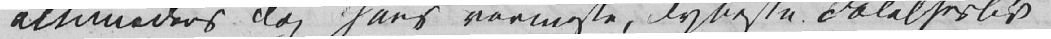altimedens dog hans varmeste, dybeste Følelsesliv
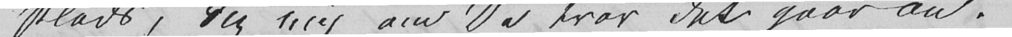Plads. Sig mig om De tror det gaar an.
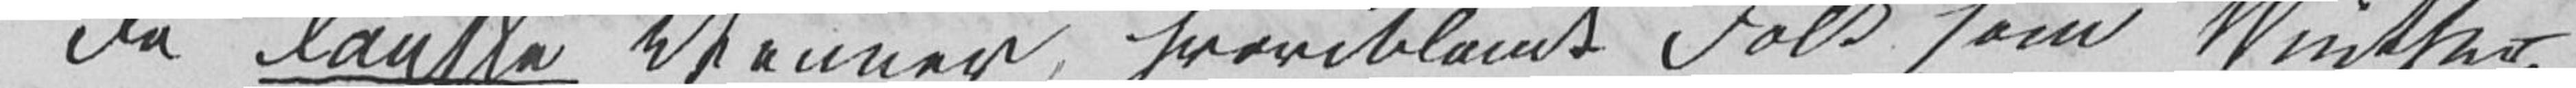de danske Venner, hvoriblandt Folk som Winther,
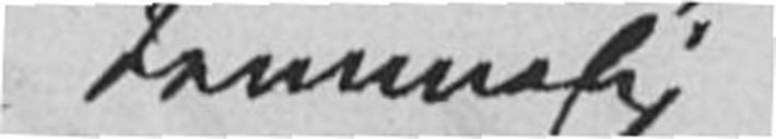temmelig
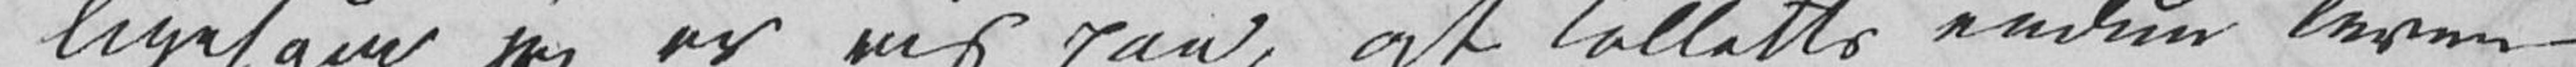ligesom jeg er vis paa, at Colletts endnu leven¬
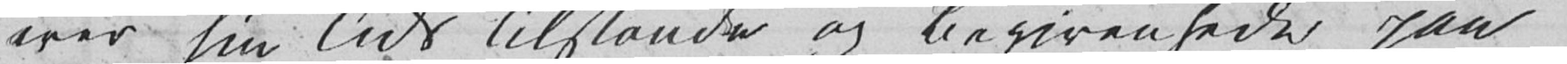over sin Tids Tilstande og Begivenheder paa
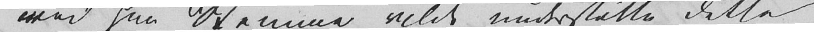med sin Stemme vilde understøtte dette
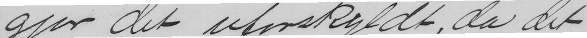gjør det uforskyldt, da det
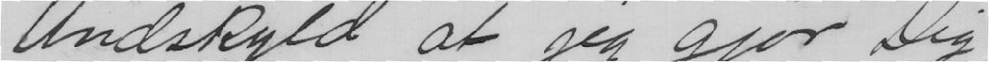Undskyld at jeg gjør Dig
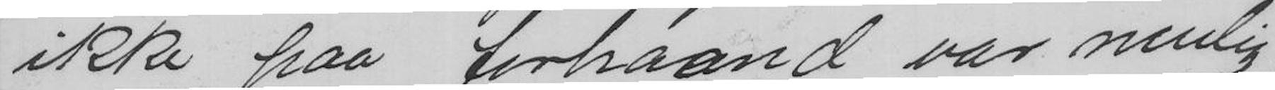ikke paa forhaand var mulig
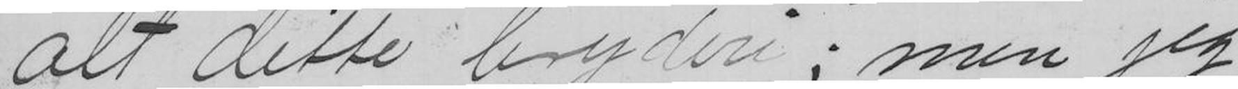alt dette bryderi; men jeg
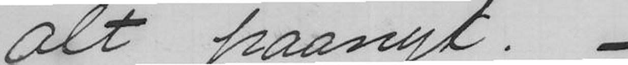alt paanyt. -
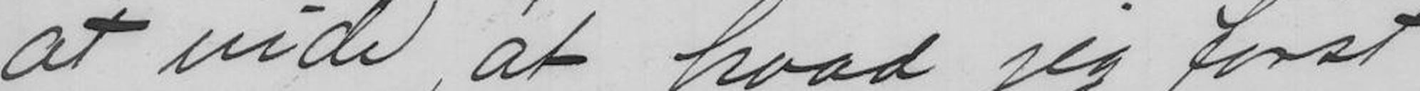at vide, at hvad jeg først
